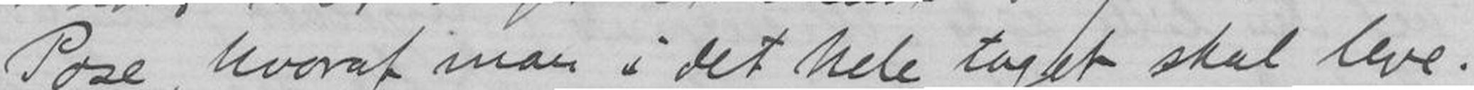Pose, hvoraf man i det hele taget skal leve.
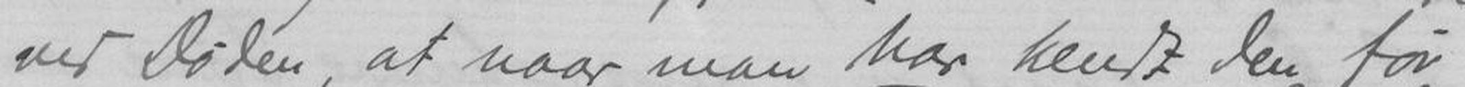ved Døden, at naar man har kendt den før
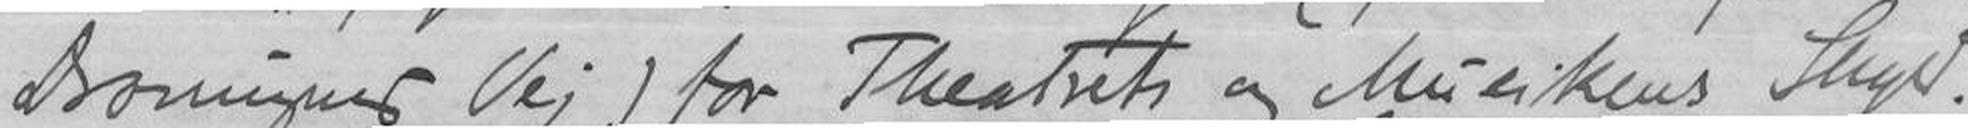Droningens Vej) for Theatrets og Musikens Skyld.
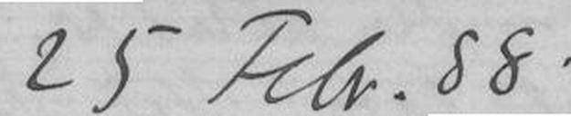25 Febr. 88
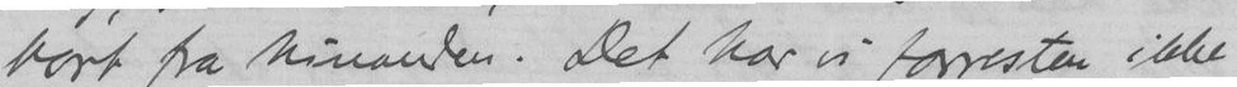bort fra hindanden. Det har vi forresten ikke
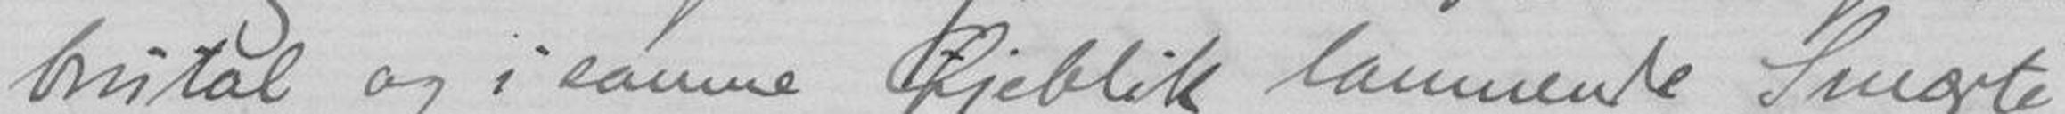brutal og samme Øjeblik lammende Smærte
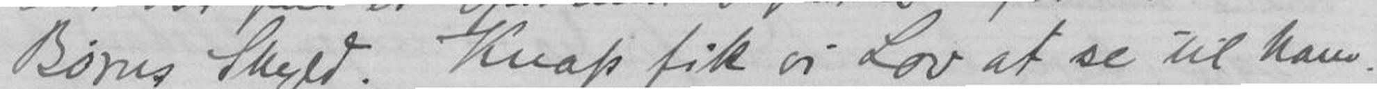Børnes Skyld. Knap fik vi Lov at se til ham.
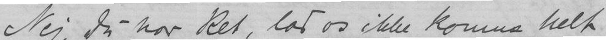Nej, du har Ret, lad os ikke komme helt
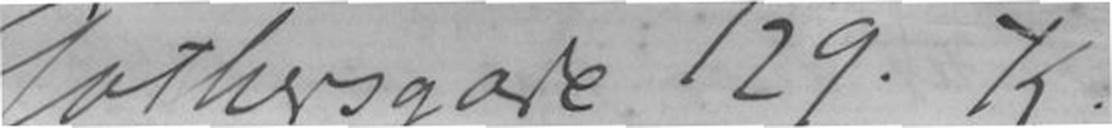Gothersgade 129 K
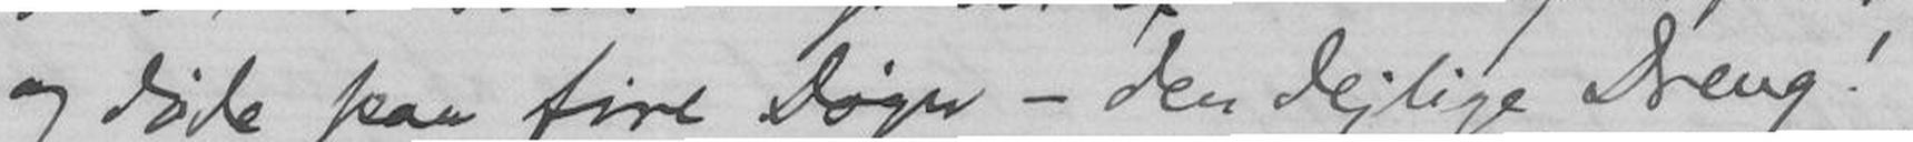og døde paa fire Døgn - den dejlige Dreng!
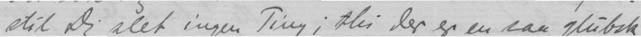stil Dig slet ingen Ting; thi der er en saa glubsk
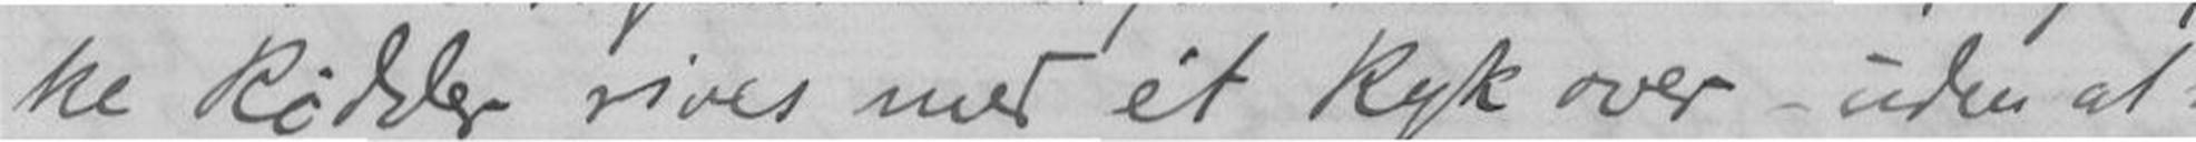ke Rødder rives med et Ryk over - uden at
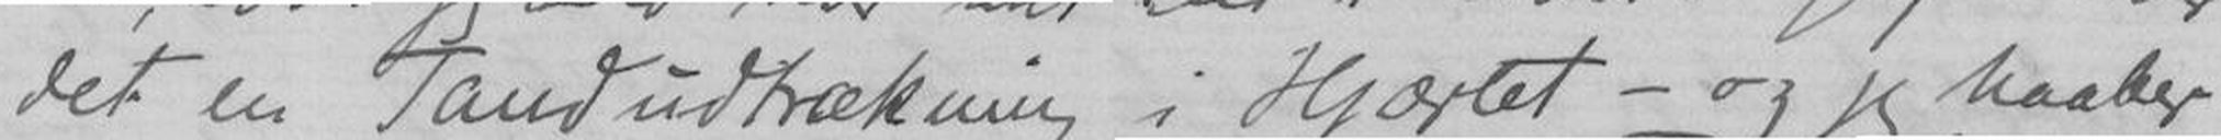det en Tandudtrækning i Hjærtet - og jeg haaber
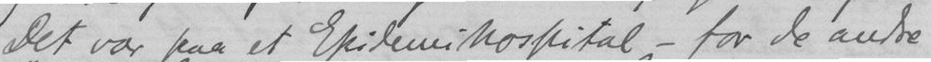Det var paa et Epidemihospital - for de andre
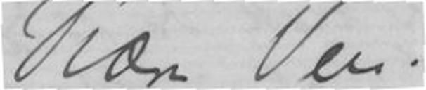Kære Ven.
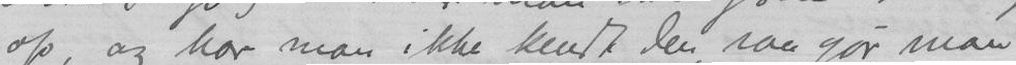op, og har man ikke kendt den, saa gør man
